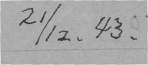21/12. 43.
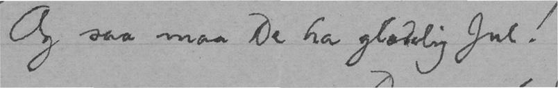Og saa maa De ha glædelig Jul!
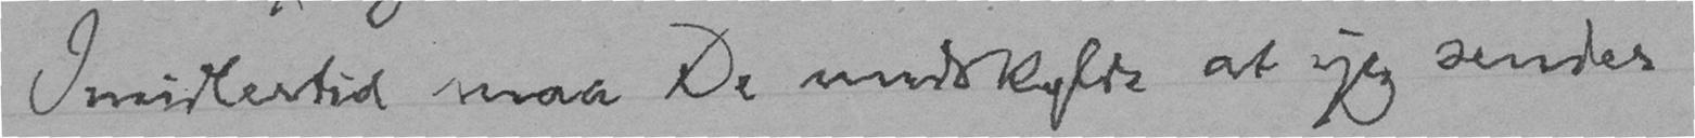Imidlertid maa De undskylde at jeg sender
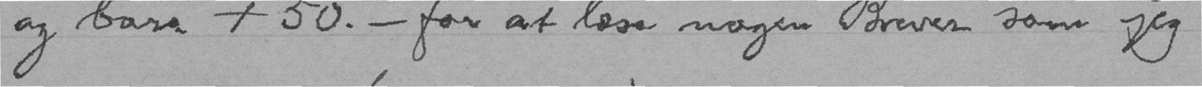og bare + 50. - for at læse nogen Brever som jeg
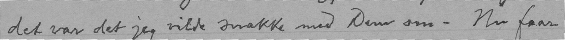det var det jeg vilde snakke med Dem om. Nu faar
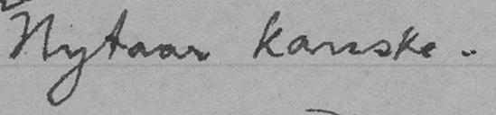Nytaar kanske.
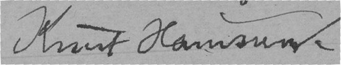Knut Hamsun.
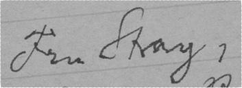Fru Stray,
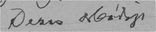Deres ærbødige
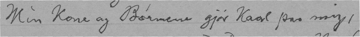Min Kone og Børnene gjør Kaal paa mig,
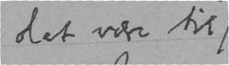det være til
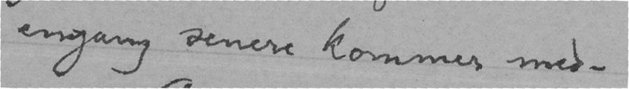engang senere kommer med.
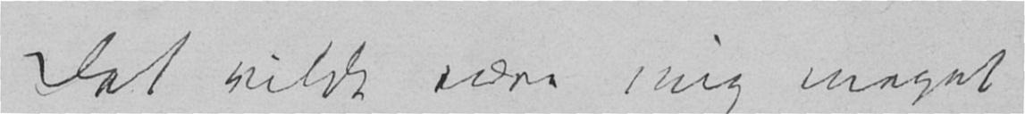Det vilde være mig meget
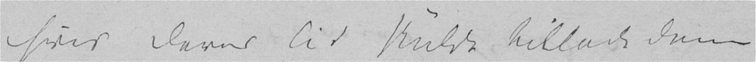hvis Deres Tid skulde tillade Dem
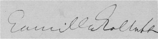Camilla Collett
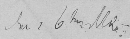Den 6te Mai.
